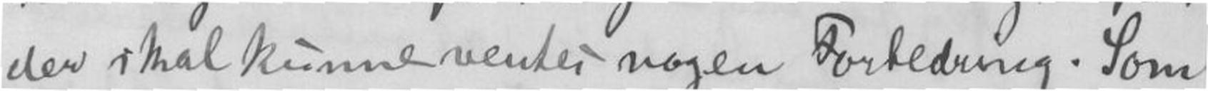der skal kunne ventes nogen Forbedring. Som
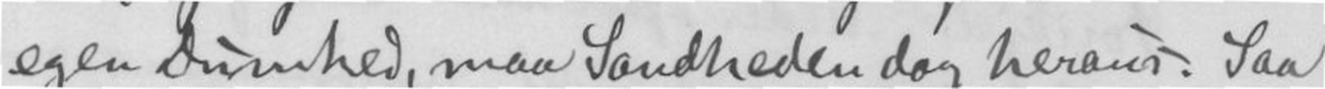egen Dunhed, men Sandheden dog heraus?. Saa
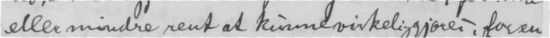eller mindre rent at kunne virkeliggjøres, for en
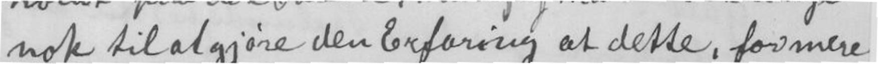nok tilat gjøre den Erfaring at dette, for mere
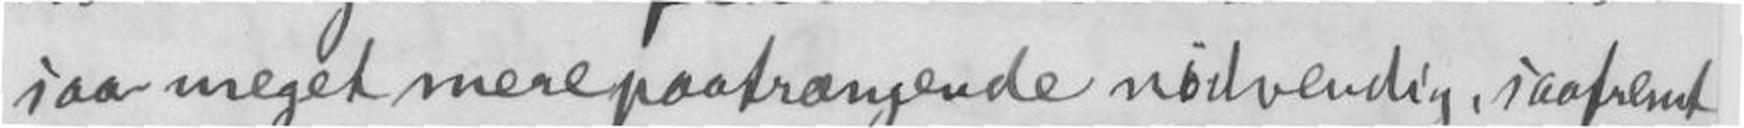saa meget mere paatrængende nødvendig, saafremt
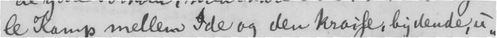le Kamp mellem Ide og den krasse, bydende, u-
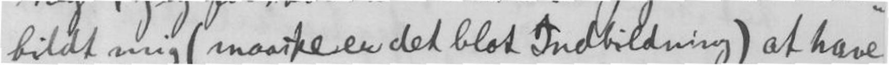bildt mig (maaske er det blot Indbilding) at have
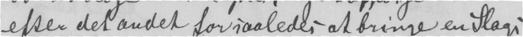efter det andet for saaledes at bringe en Slags
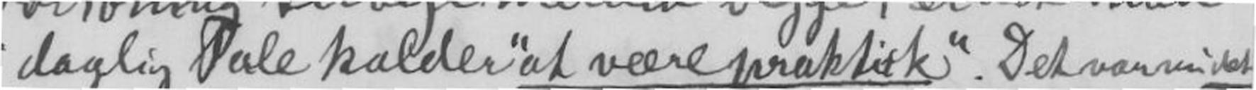i daglig Tale kalder "at være praktisk". Det var nu det.
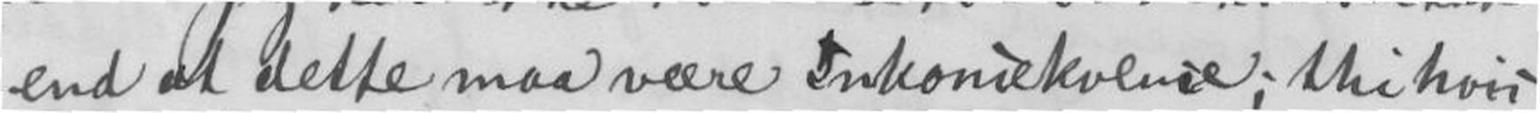end at dette maa være Inkonsekvense; thi hvori
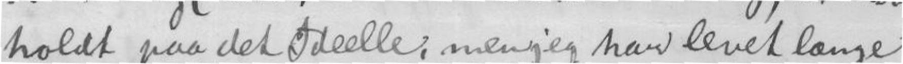holdt paa det ideelle, men jeg har levet længe
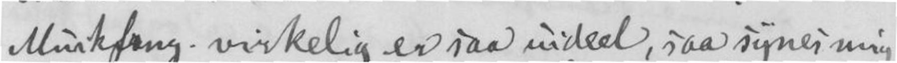Musikfrng. virkelig er saa uideel, saa synes mig
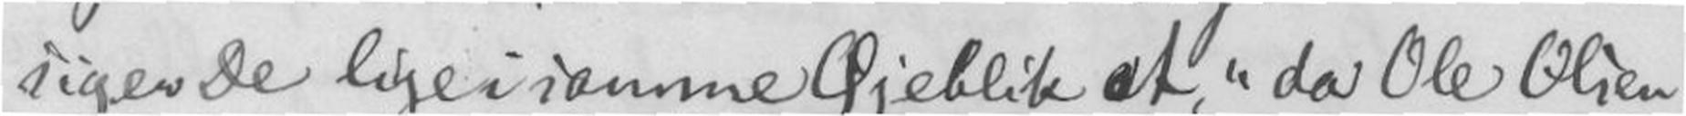siger De lige i samme Øjeblik at, "da Ole Olsen
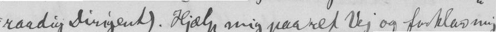raadig Dirigent). Hjælp mig paa ret Vej og forklar mig
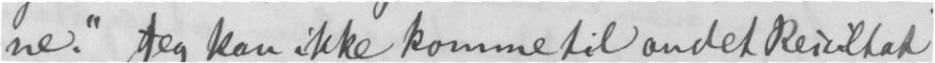ne". Jeg kan ikke komme til andet Resultat
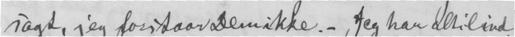sagt, jeg forstaar Dem ikke. - Jeg har altid ind-
